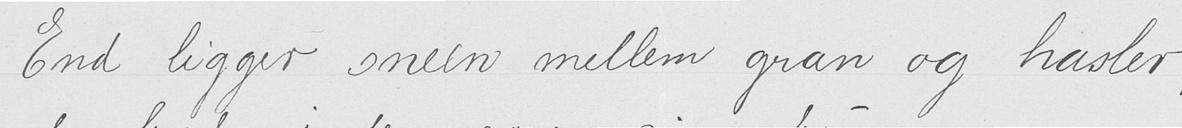End ligger sneen mellem gran og hasler,
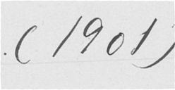(1901)
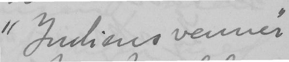"Indiens venner"
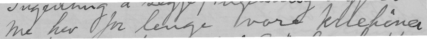Me hev for lenge vore knehöner
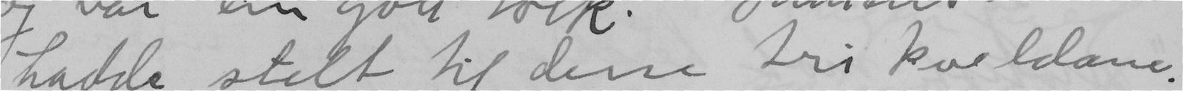hadde stelt til desse tri kveldane.
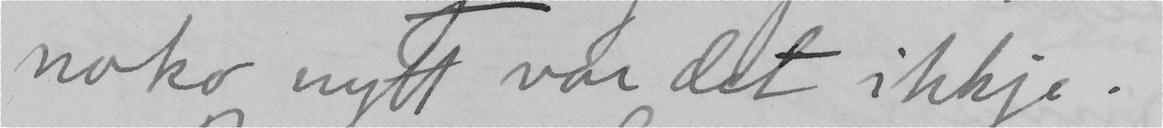noko nytt var det ikkje.
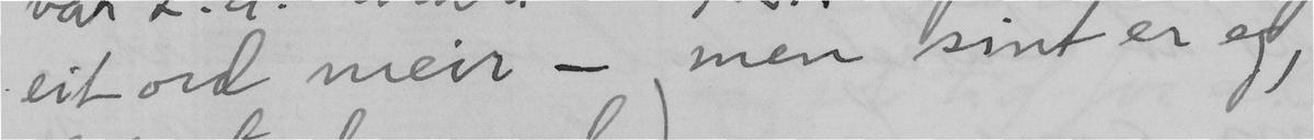eit ord meir – men sint er eg
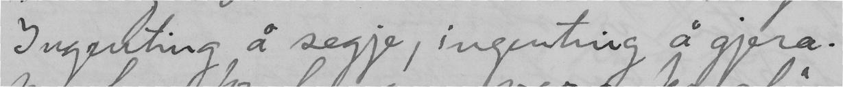Ingenting å segje, ingenting å gjera.
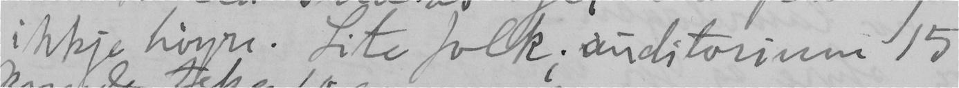ikkje höyre. Lite folk; auditorium 15
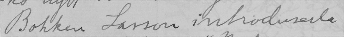Bokken Lasson introduserte
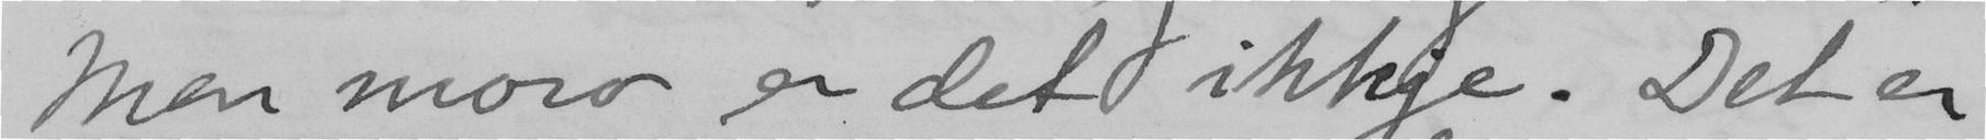Men moro er det ikkje. Det er
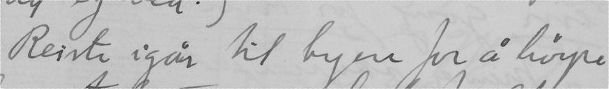Reiste igår til byen for å höyre
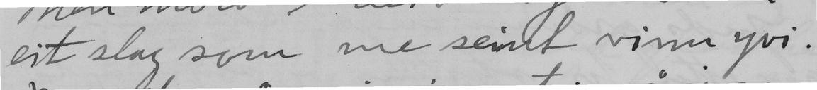eit slag som me seint vinn yvi.
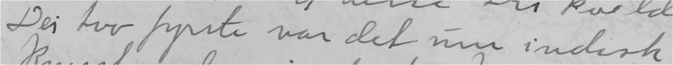Dei tvo fyrste var det um indisk
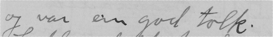og var sin god tolk.
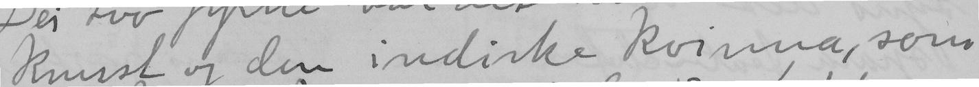kunst og den indiske kvinna, som
